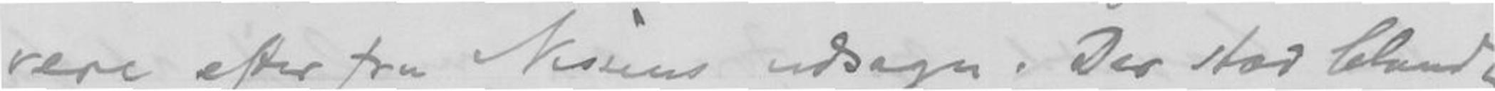vere efter fru Nissens udsagn. Der stod blandt
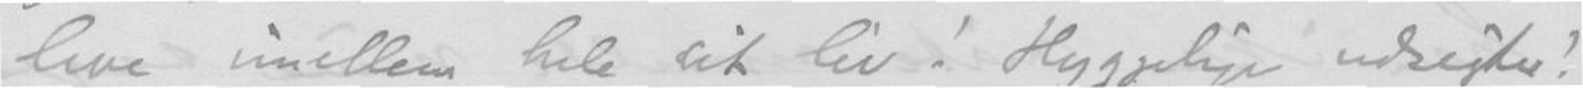leve imellem hele sit liv! Hyggelige udsigter!
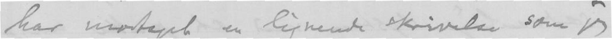har modtaget en lignende skrivelse som jeg
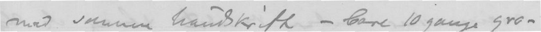med samme håndskrift - bare 10 gange gro-
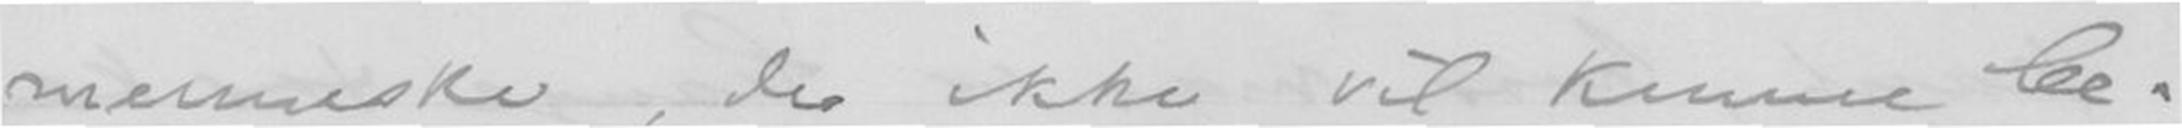menneske, der ikke vil kunne be-
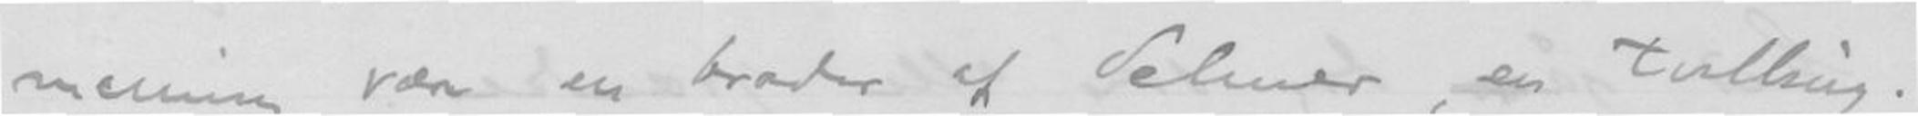mening være en broder af Selmer, en tulling.
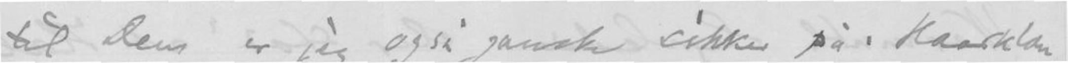til Dem er jeg også ganske sikker på. Haarklou
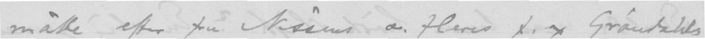måtte efter fru Nissen o. fleres f.x. Grøndahls
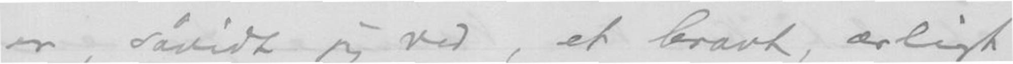er, såvidt jeg ved, et bravt, ærligt
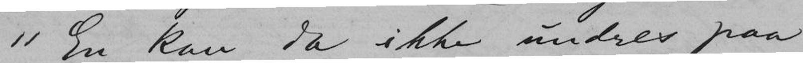"En kan da ikke undres paa
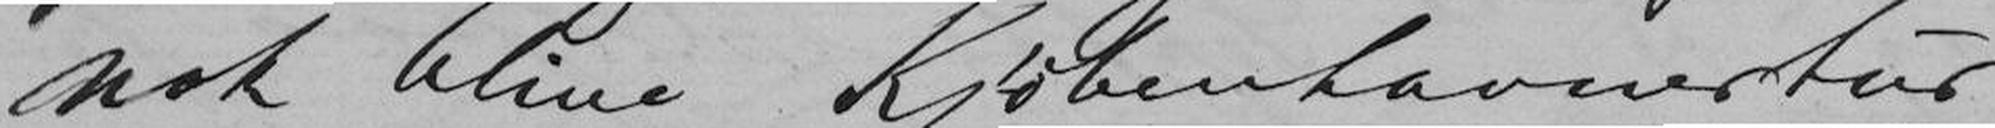nok blive Kjøbenhavnertur
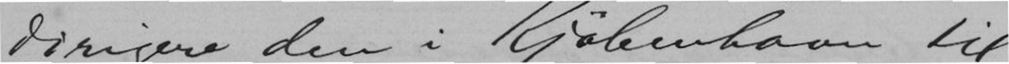dirigere den i Kjøbenhavn til
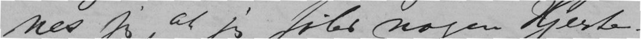nes jeg, at jeg føler nogen Hjerte-
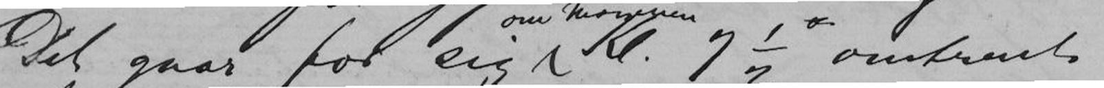Det gaar for sig om Morgenen Kl. 7 ½ omtrent.
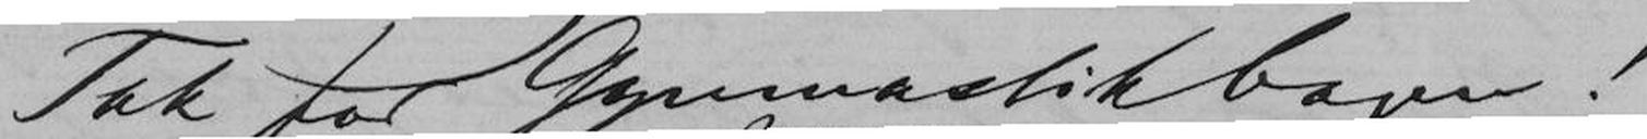Tak for Gymnastikbogen!
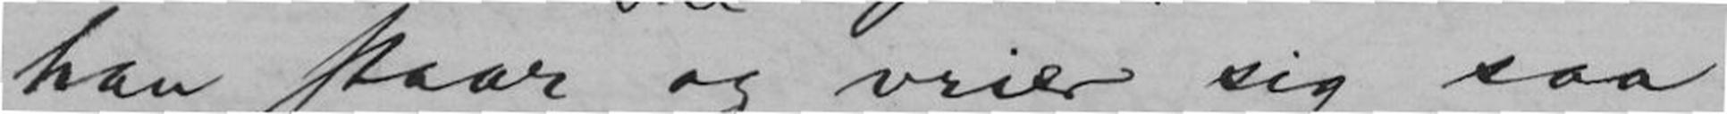han staar og vrier sig saa
